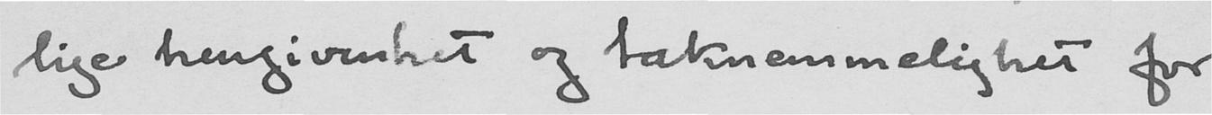lige hengivenhet og taknemmelighet for
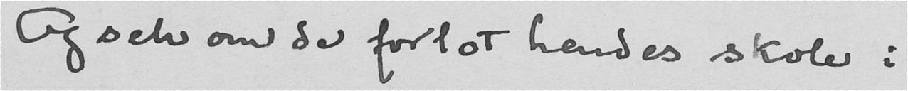Og selv om de forlot hendes skole i
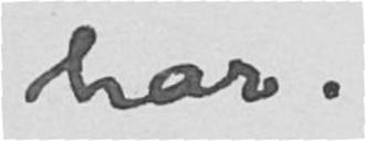har.
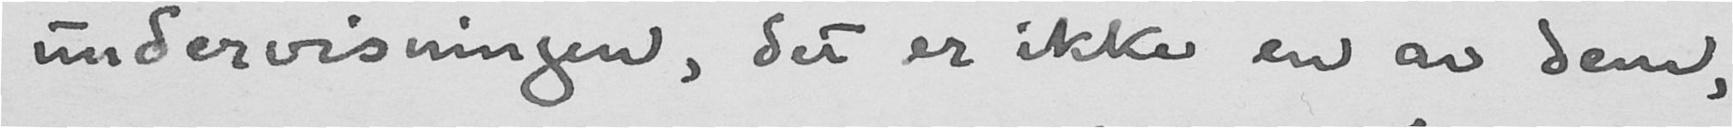undervesningen, det er ikke en av dem,
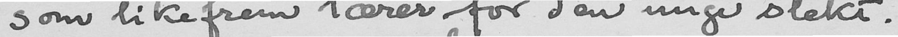som likefrem lærer for den unge slekt.
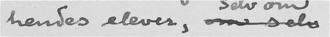hendes elever, om selv
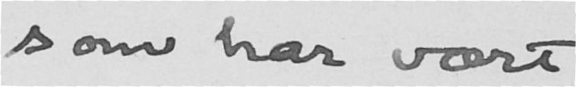som har vært
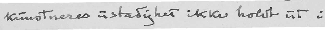kunstneres ustadighet ikke holdt ut i
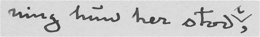ning hun her stod,
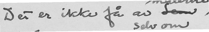Det er ikke få av dem
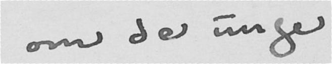om de unge
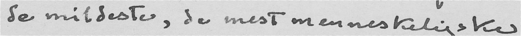de mildeste, de mest menneskeligske
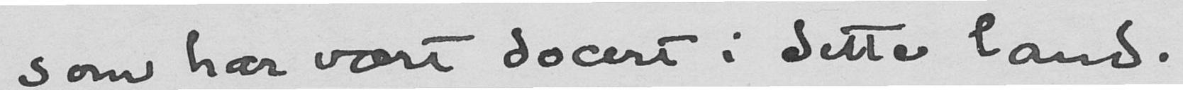som har vært docert i dette land.
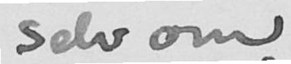selv om
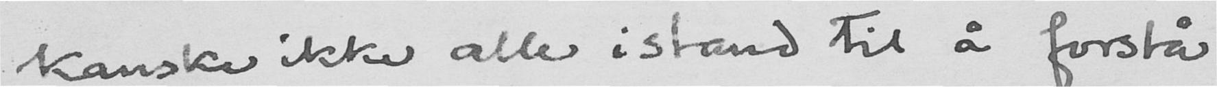kanske ikke alle istand til å forstå
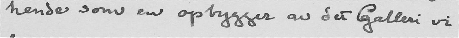hende som en opbygger av det Galleri vi
